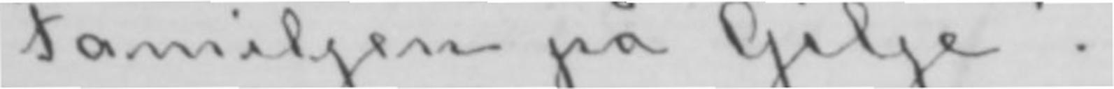og «Familjen på Gilje».
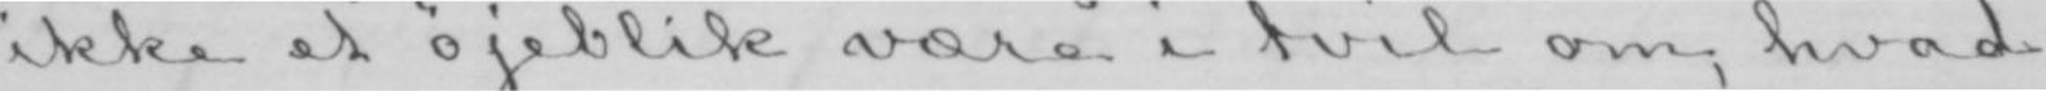ikke et øjeblik være i tvil om, hvad
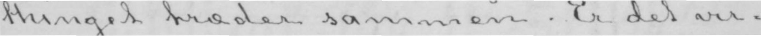thinget træder sammen. Er det vir-
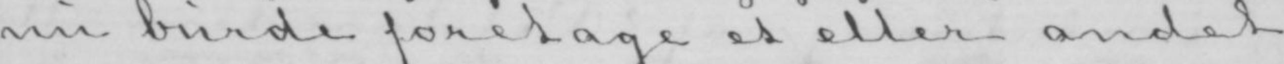nu burde foretage et eller andet
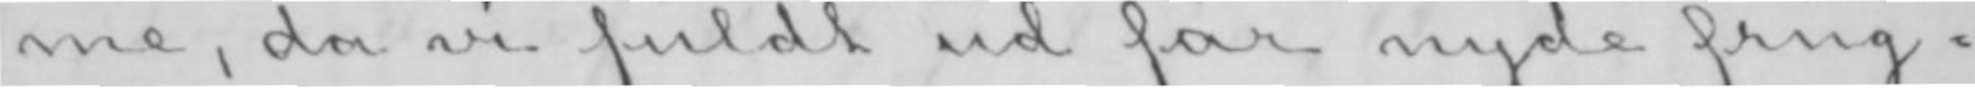me, da vi fuldt ud får nyde frug-
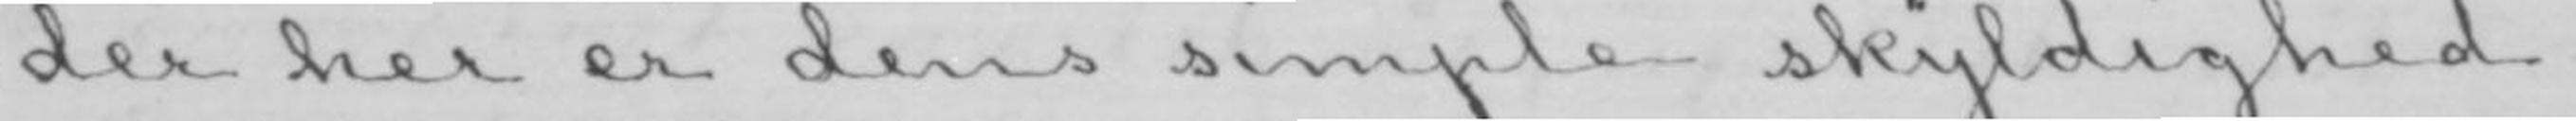der her er dens simple skyldighed.
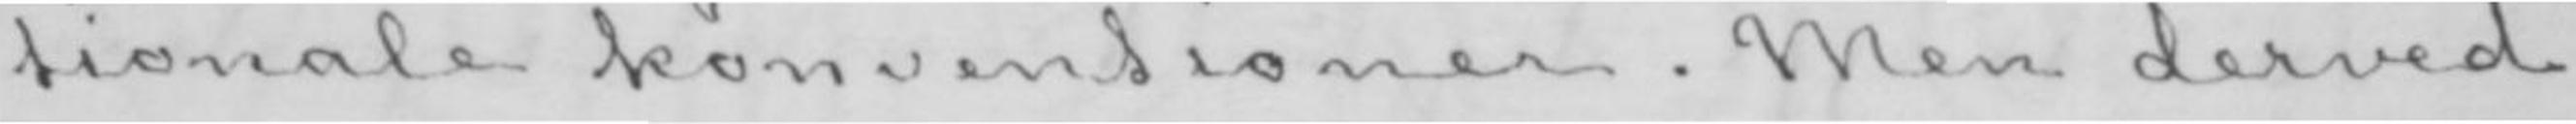tionale konventioner. Men derved
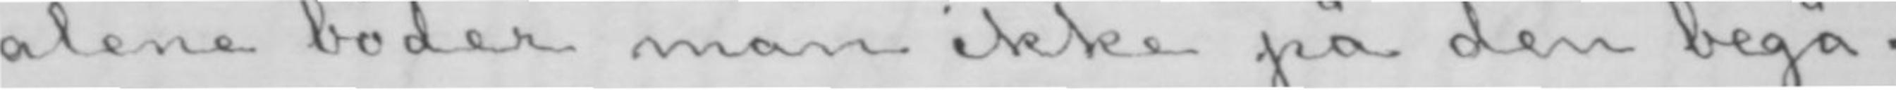alene bøder man ikke på den begå-
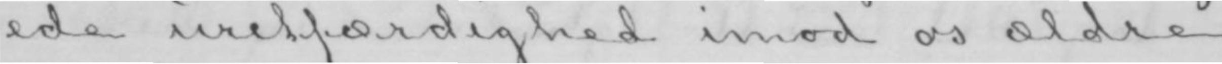ede uretfærdighed imod os ældre
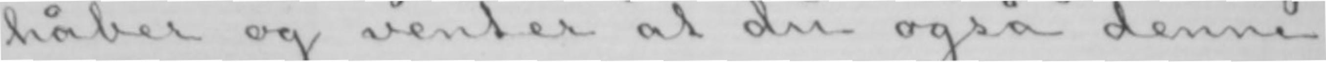håber og venter at du også denne
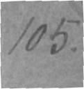105.
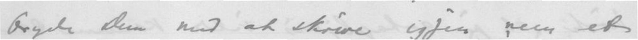bryde Dem med at skrive igjen, men et
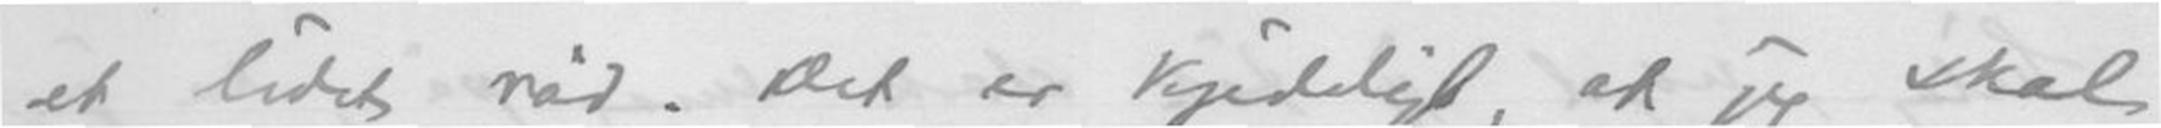et lidet råd. Det er kjedeligt, at jeg skal
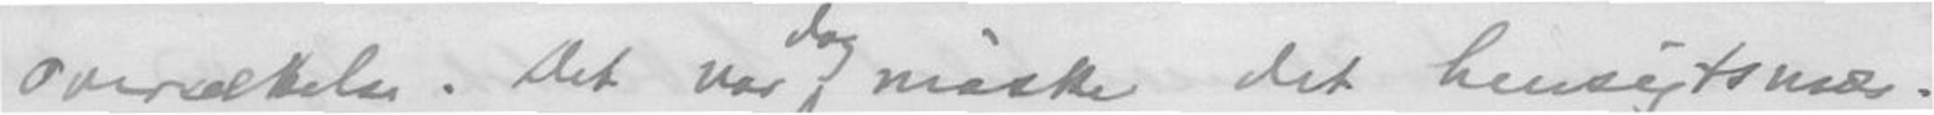overraskelse. Det var dog måske det hensigmæs-
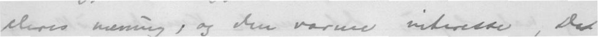deres mening, og den varme intresse, Det
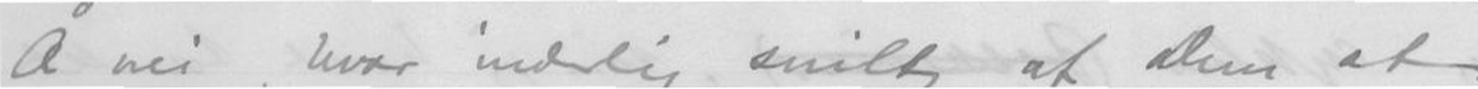Å nei, hvor inderlig snilt af Dem at
